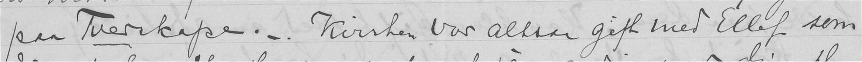paa Tverrkape. - Kirsten var altsaa gift med Ellef som
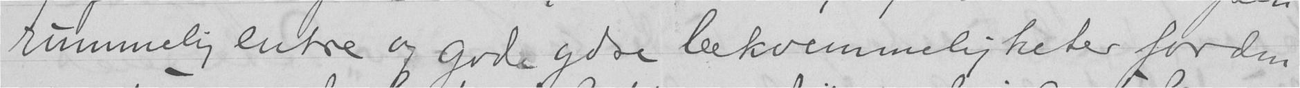rummelig entre og gode gode bekvemmeligheter for den
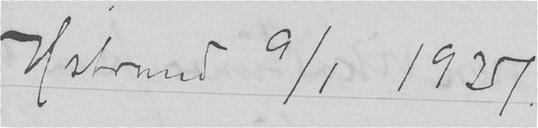Hstrand 9/1 1925.
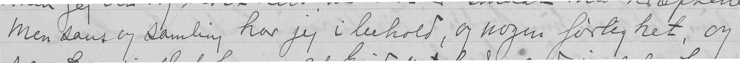Men sans og samling har jeg i behold, og nogen førlighet, og
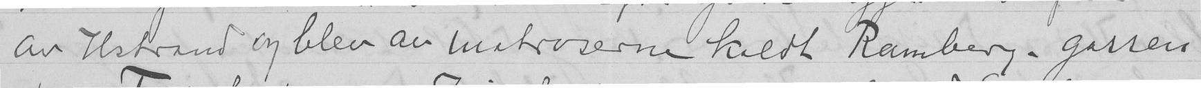av Hstrand og blev av matroserne kaldt Ramberg-gassen
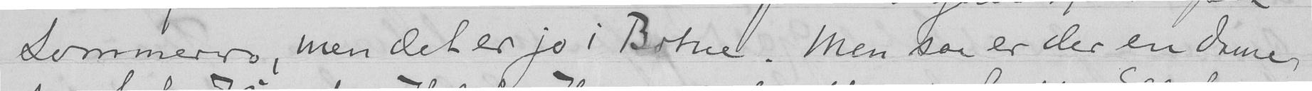Sommerro, men det er jo i Botne. Men saa er der en dame,
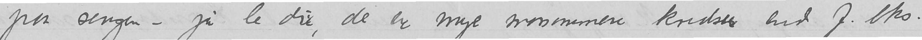paa sengen – ja le du, de er mye mosommere kredser end f.eks.
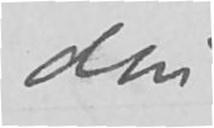din
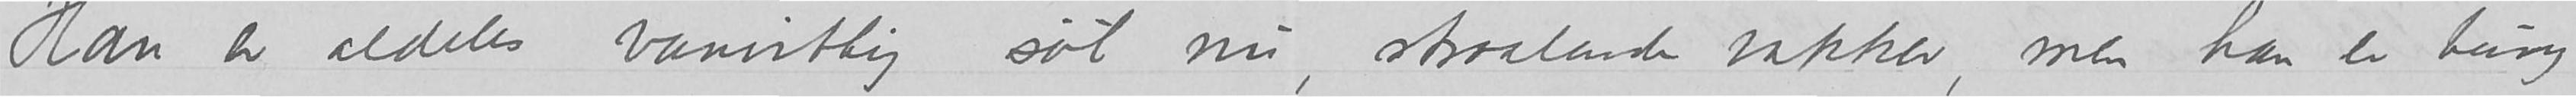Han er aldeles vanvittig søt nu, straalende vakker, men han er tung
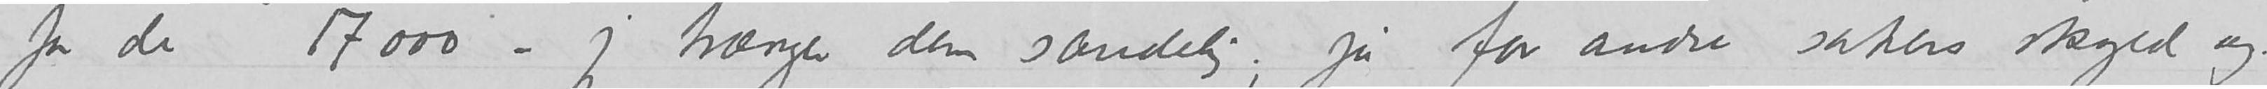for de 17 000 – jeg trænger dem sandelig; ja for andre sakers skyld og.
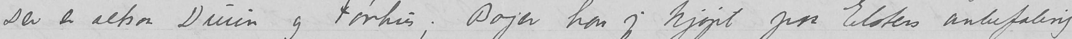Der er altsaa Duun og Fønhus;, Bojer har jeg kjøpt paa Elsters
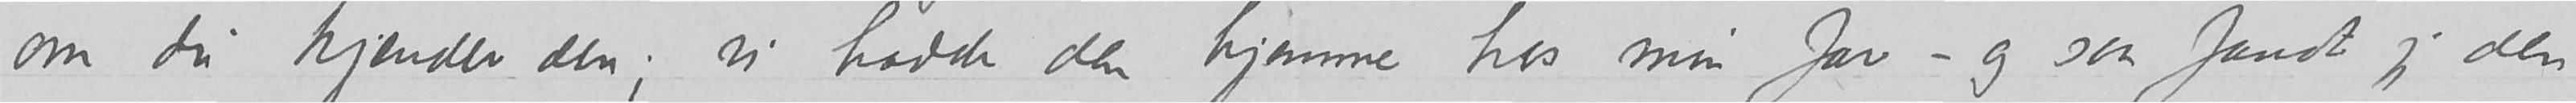om du kjender den; vi hadde den hjemme hos min far – og saa fandt jeg den
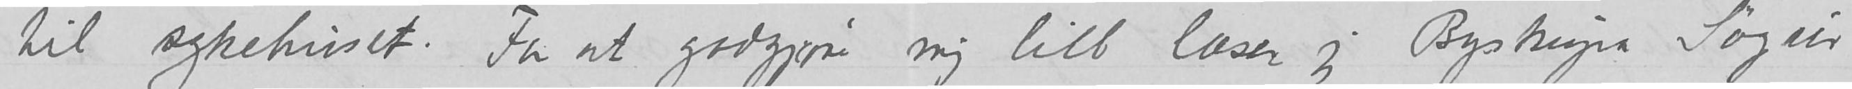til sykehuset. For at godgjøre mig litt læser jeg  Byskupa Søgur
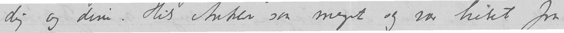dig og dine. Hils Anker saa meget og saa vær hilset fra
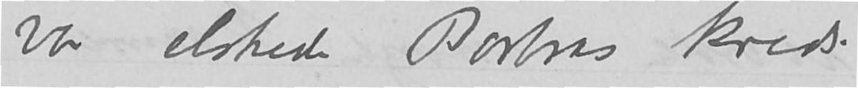vaar elskede Barbras kreds.
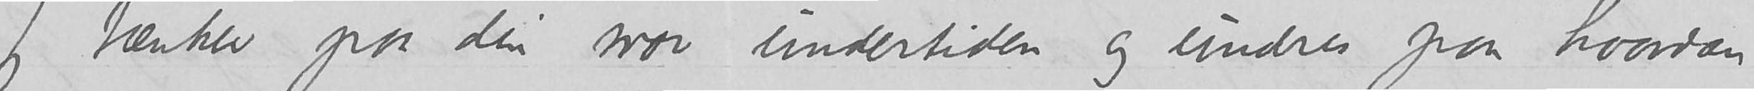Jeg tænker paa din mor undertiden og undres paa hvordan
